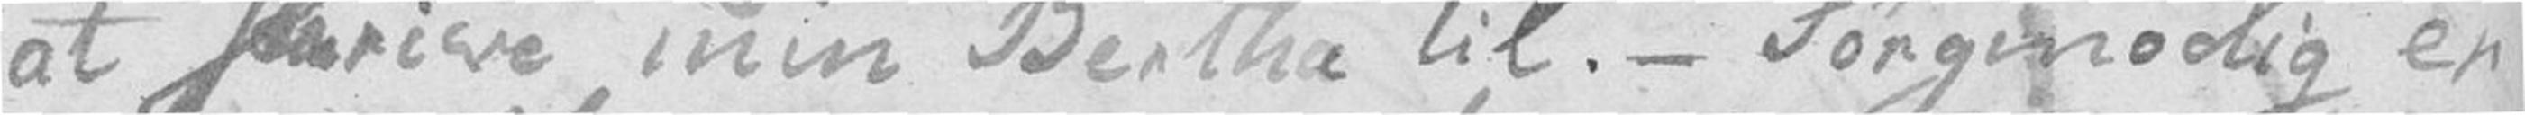at skrive min Bertha til. – Sørgmodig er
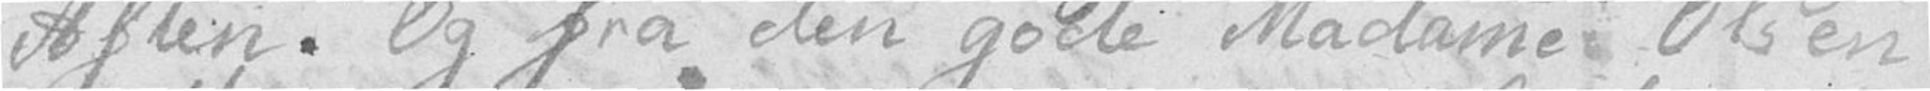Aften. Og fra den gode Madamme Olsen
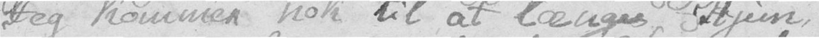Jeg kommer nok til at længes Hjem,
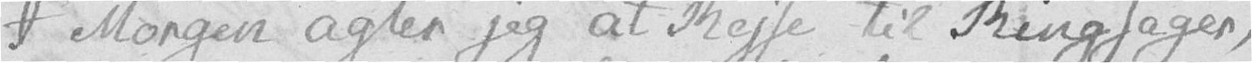I Morgen agter jeg at Rejse til Ringsager,
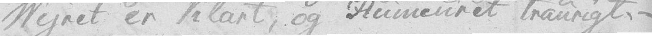Vejret er Klart, og Humeuret traurigt. –
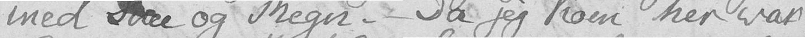med Snee og Regn. – Da jeg kom her var
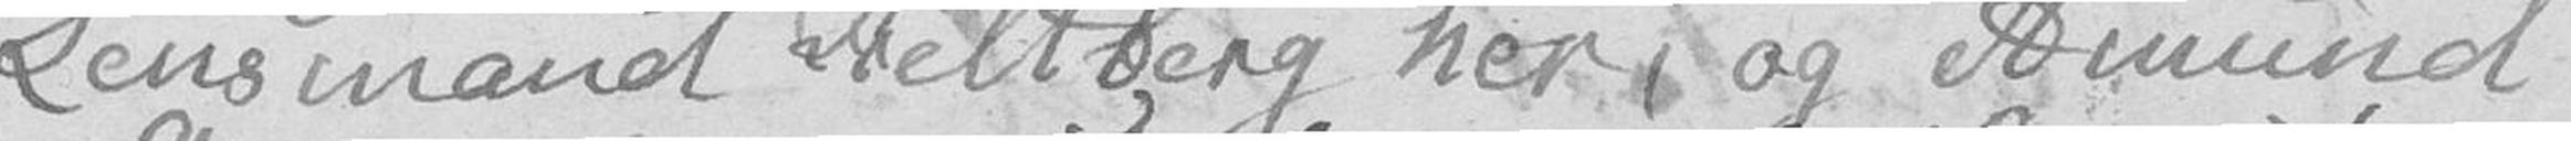Lensmand Heltberg her og Amund
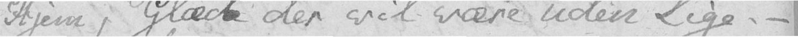Hjem, Glæde der vil være uden Lige. –
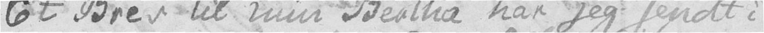Et Brev til min Bertha har jeg sendt i
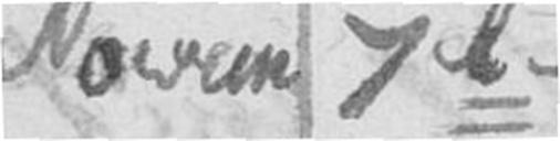Novem 7de
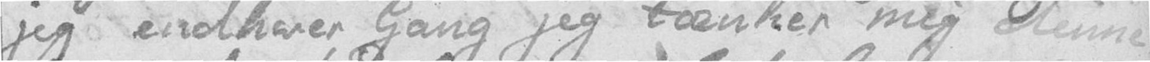jeg endhver Gang jeg tænker mig denne
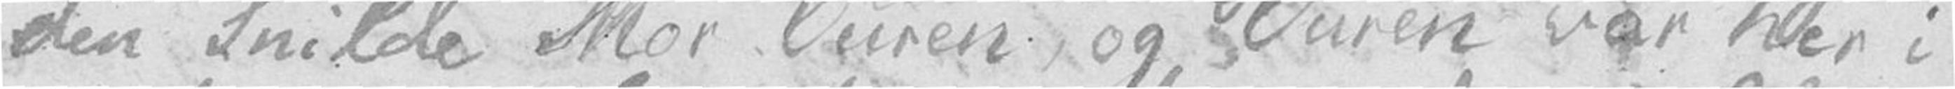den Snilde Mor Ouren, og Ouren var her i
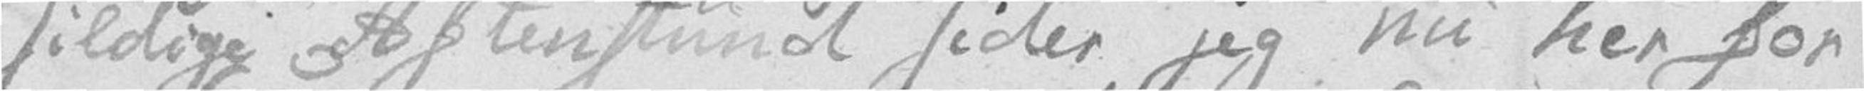sildige Aftenstund sider jeg nu her for
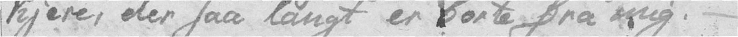Kjere, der saa langt er borte fra mig. –
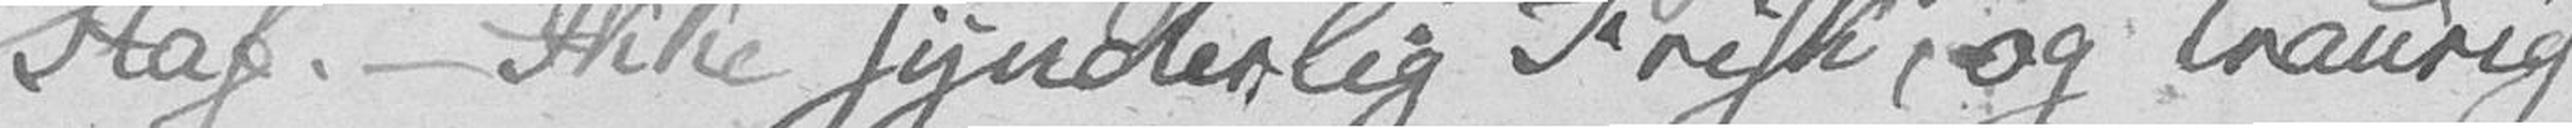Staf. – Ikke synderlig Frisk, og traurig
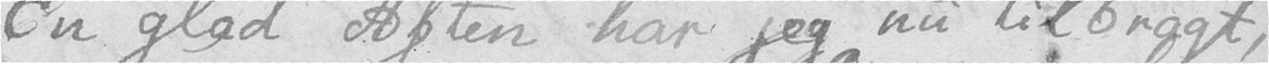En glad Aften har jeg nu tilbragt,
